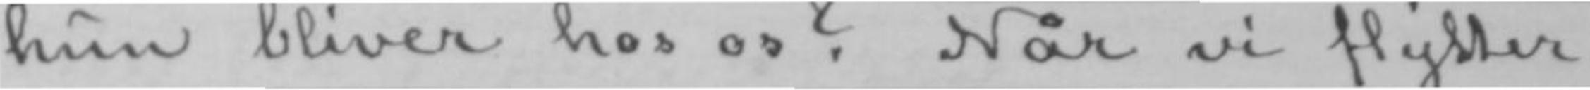hun bliver hos os? Når vi flytter
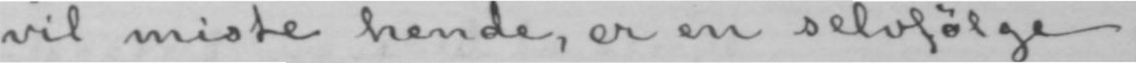vil miste hende, er en selvfølge;
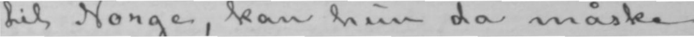til Norge, kan hun da måske
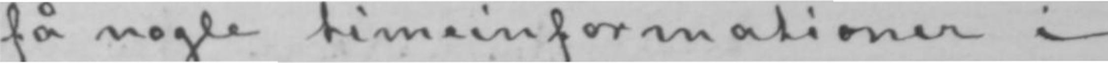få nogle timeinformationer i
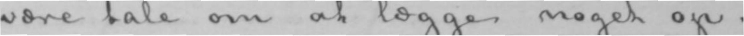være tale om at lægge noget op.
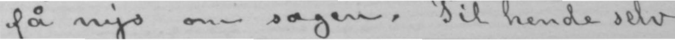få nys om sagen. Til hende selv
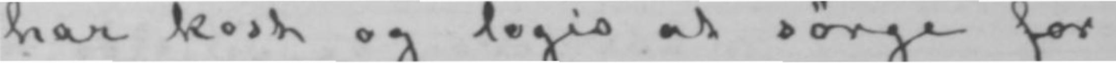har kost og logis at sørge for,
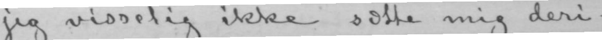jeg visselig ikke sætte mig deri-
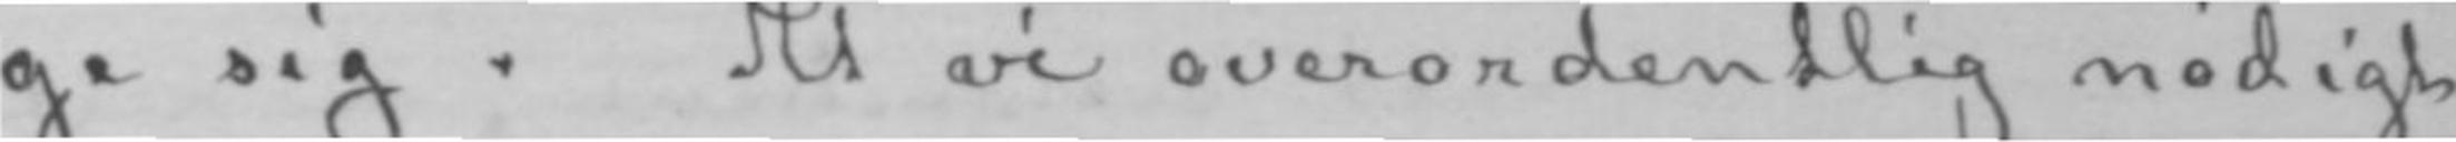ge sig. At vi overordentlig nødigt
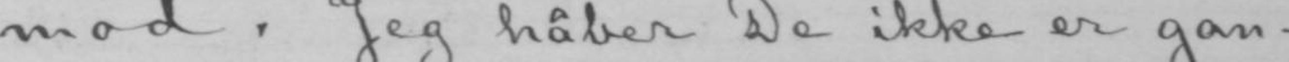mod. Jeg håber De ikke er gan-
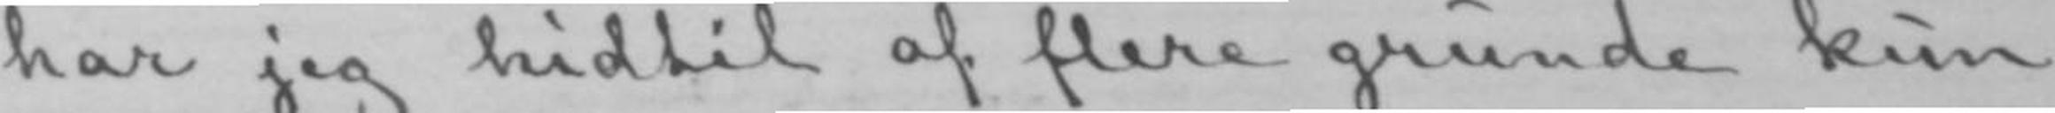har jeg hidtil af flere grunde kun
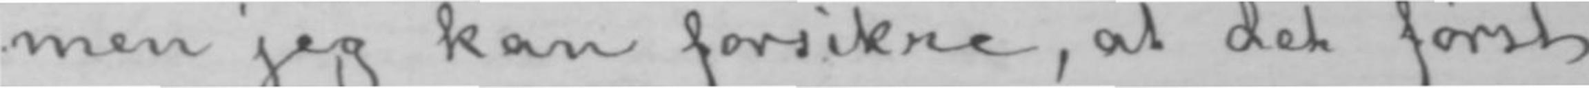men jeg kan forsikre, at det først
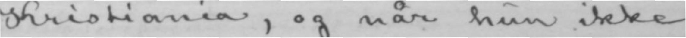Kristiania, og når hun ikke
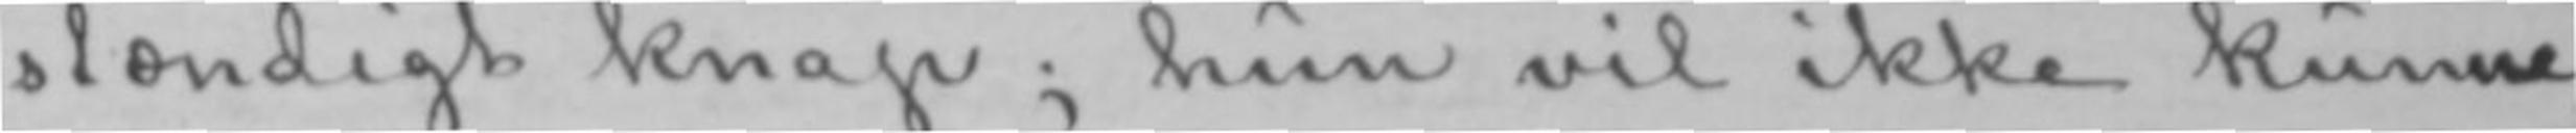stændigt knap; hun vil ikke kunne
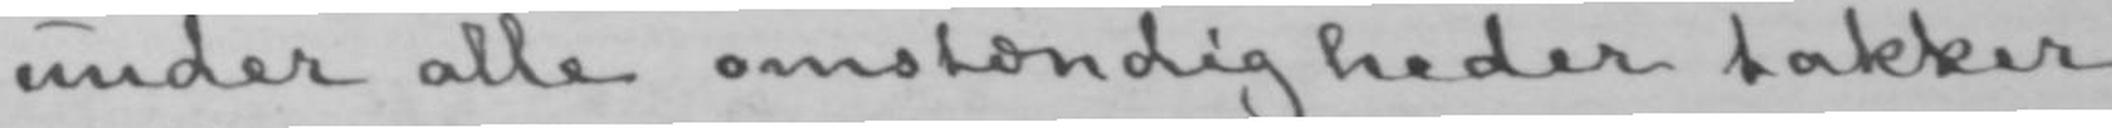under alle omstændigheder takker
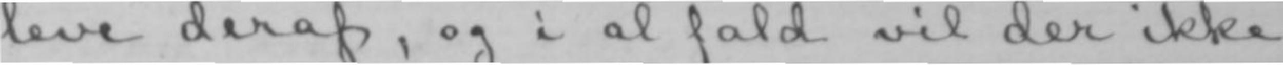leve deraf, og i al fald vil der ikke
